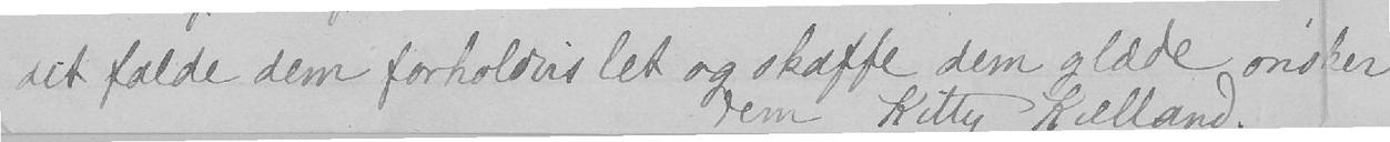det falde dem forholdvis let og skaffe dem glæde ønsker
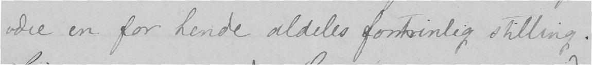være en for hende aldeles fortrinlig stilling.
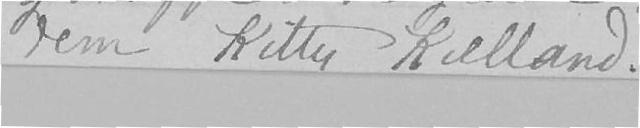dem Kitty Kielland.
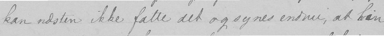kan næsten ikke fatte det og synes endnu, at han
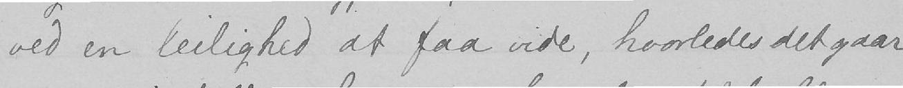ved en leilighed at faa vide, hvorledes det gaar
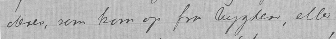deres, som kom op fra bygden, eller
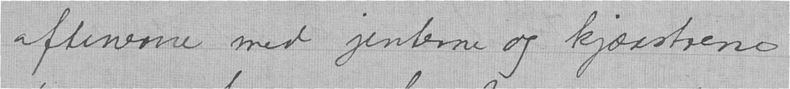aftenerne med jenterne og kjærestrene
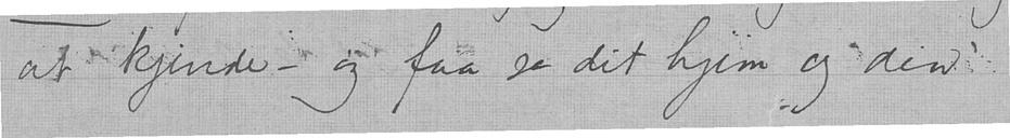at kjende — og faa se dit hjem og din
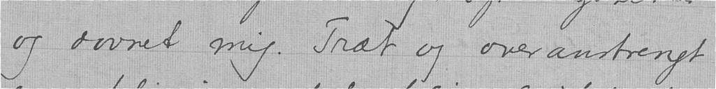og dovnet mig. Træt og overanstrengt
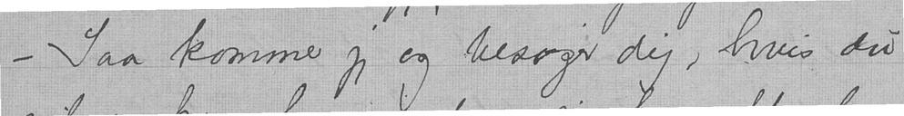— Saa kommer jeg og besøger dig, hvis du
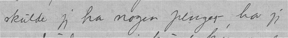skulde jeg ha nogen penger, har jeg
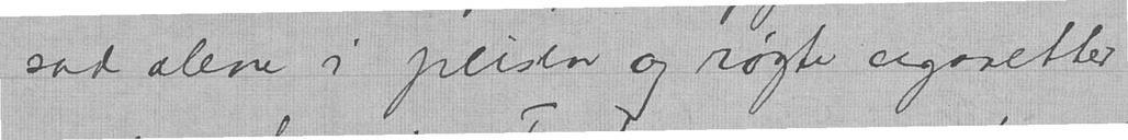sad alene i peisen og røgte cigaretter
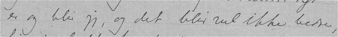er og blir jeg, og det blir vel ikke bedre,
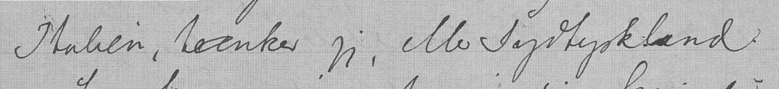Italien, tænker jeg, eller Sydtyskland.
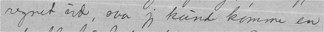regnet ud, saa jeg kunde komme en
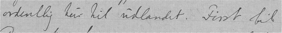ordentlig tur til udlandet. Først til
